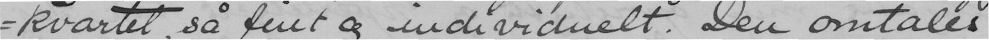=kvartet, så fint og individuelt. Den omtales
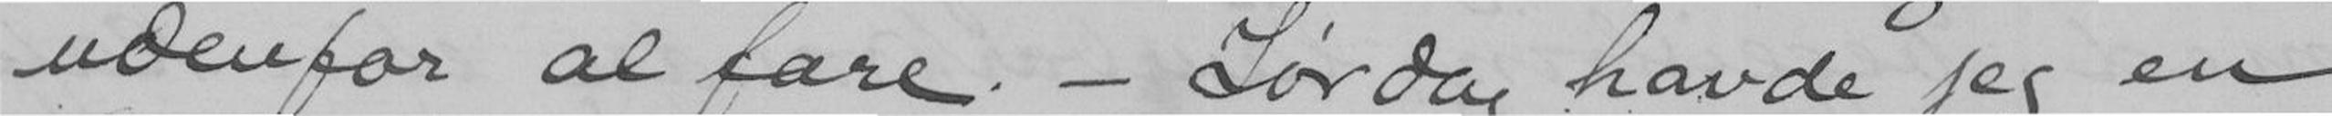udenfor al fare. - Lørdag havde jeg en
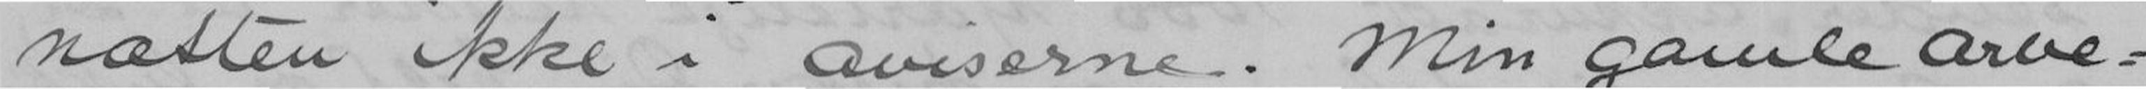næsten ikke i aviserne. Min gamle arve-
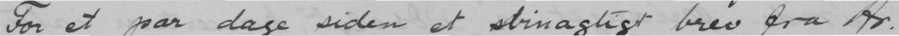For et par dage siden et svinagtigt brev fra Hr.
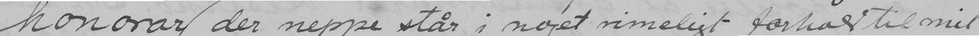honorar (der neppe står i noget rimeligt forhold til mit
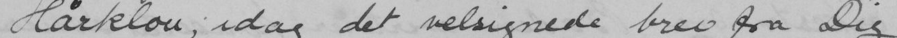Hårklou; idag det velsignede brev fra Dig.
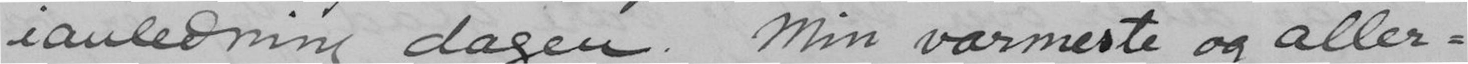ianledning dagen. Min varmeste og aller-
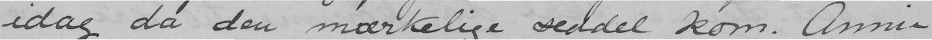idag da den mærkelige seddel kom. Annie
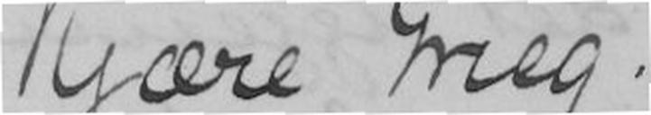Kjære Grieg!
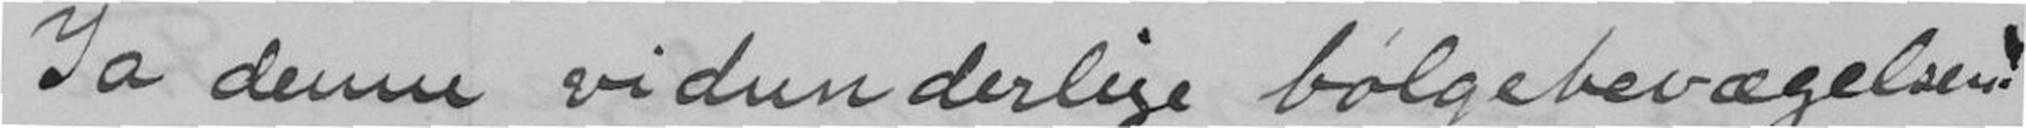Ja denne vidunderlige bølgebevægelsen!
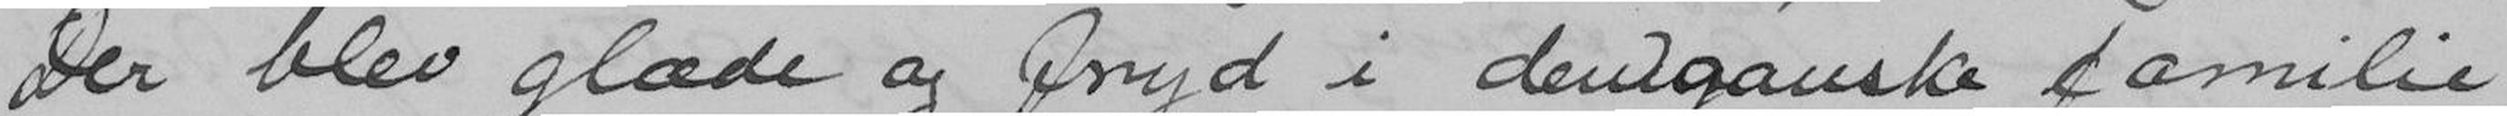Der blev glæde og fryd i den ganske familie
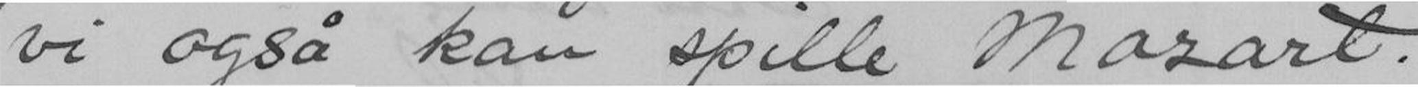vi også kan spille Mozart.
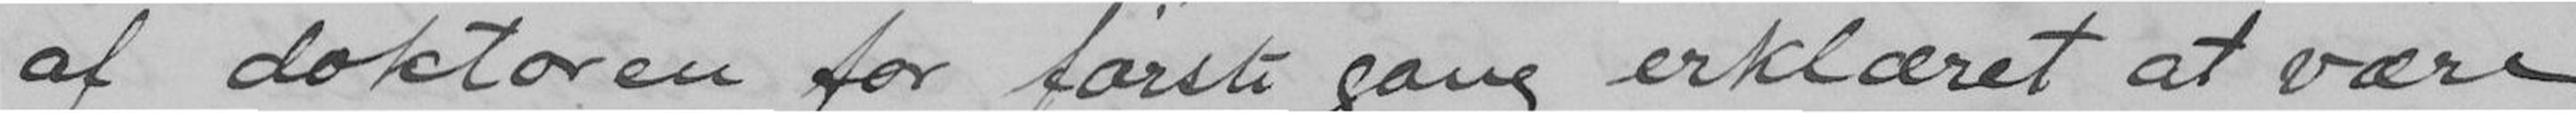af doktoren for første gang erklæret at være
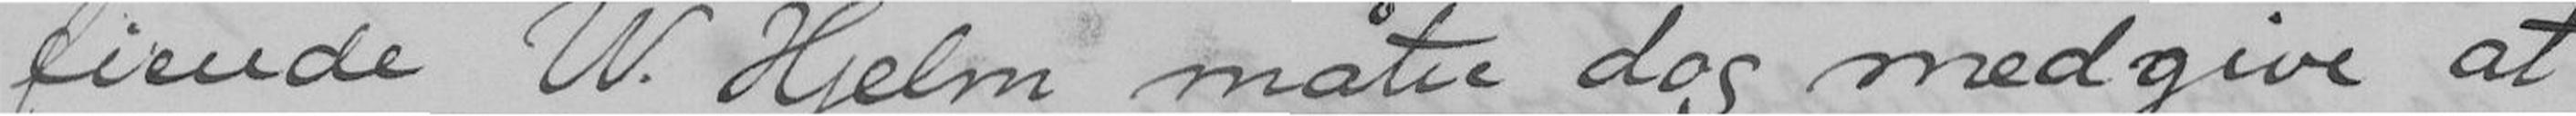fiende W. Hjelm måtte dog medgive at
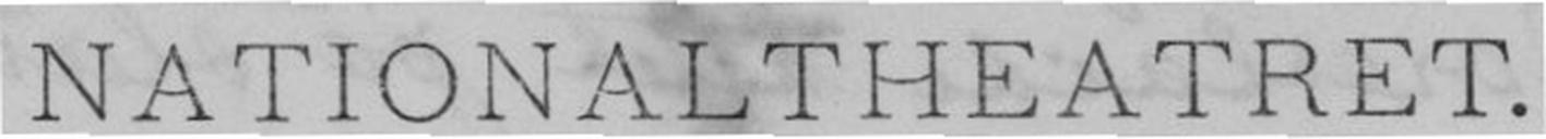NATIONALTHEATRET
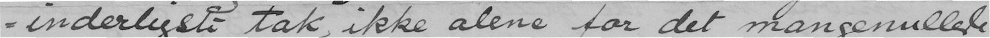inderligste tak, ikke alene for det mangenullede
